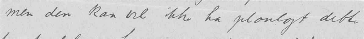- men den kan vel ikke ha planlagt dette
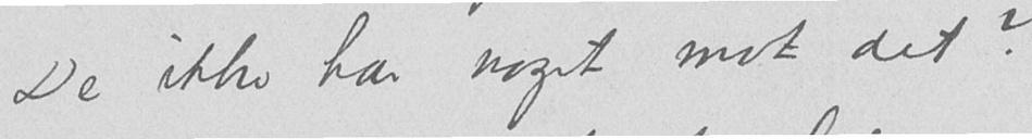De ikke har noget mot det?
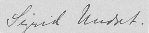Sigrid Undset.
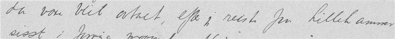da være blit avtalt, efter jeg reiste fra Lillehammer
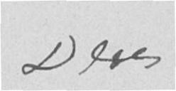Deres
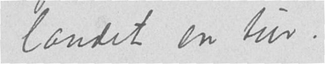landet en tur.
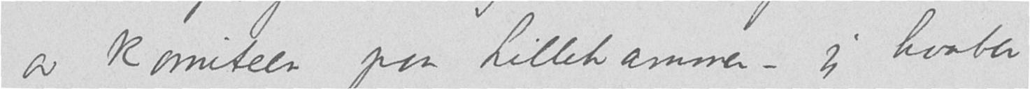av komiteen paa Lillehammer - jeg haaber
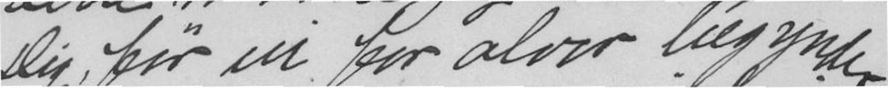Dig, før vi for alvor begynder
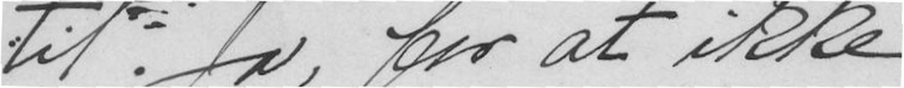til. Ja, for at ikke
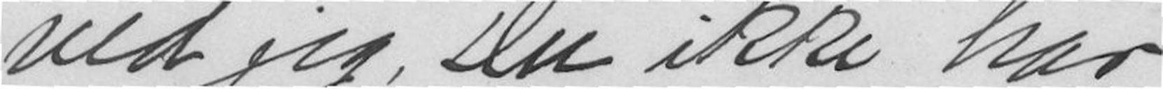ved jeg, Du ikke har
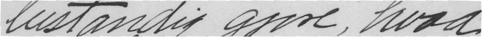bestandig gjøre, hvad
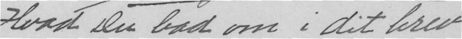Hvad Du bad om i dit brev
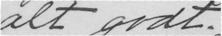alt godt.
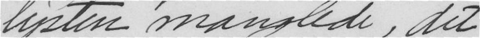lysten manglede, det
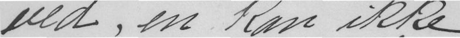ved, en kan ikke
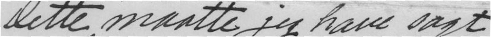Dette maatte jeg have sagt
